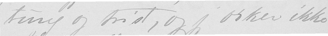tung og trist, og jeg orker ikke
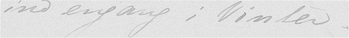ind engang i Vinter.
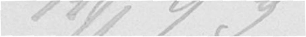14/1 93
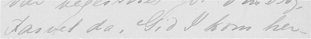Farvel da. Gid I kom her-
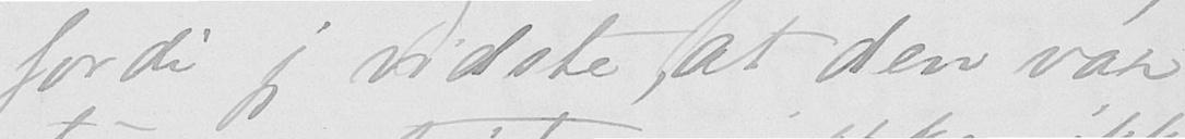fordi jeg vidste, at den var
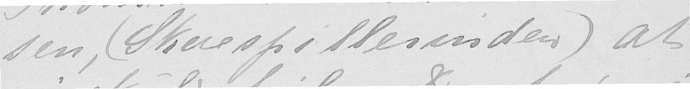sen (Skuespillerinden) at
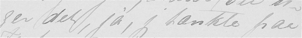ger det, ja, jeg tænkte paa
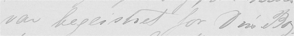var begeistret for Din Bog.
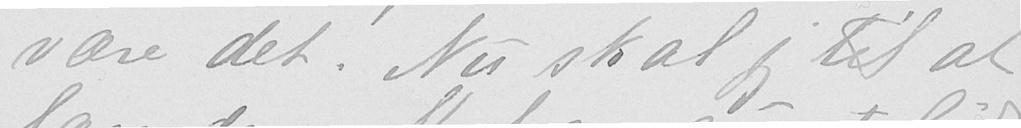være det! Nu skal jeg til at
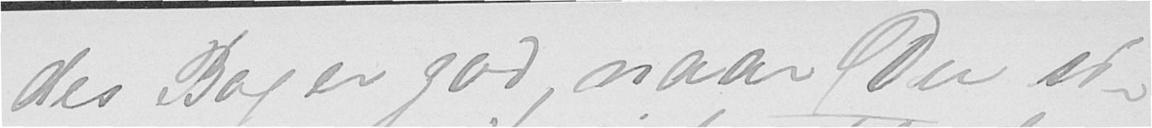des Bog er god, naar Du si-
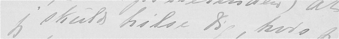jeg skulde hilse dig, hvis jeg
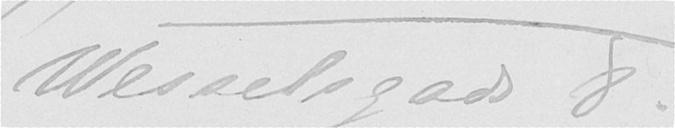Wesselsgade 8.
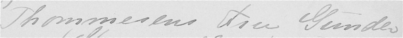Thommesens Fru Gunder-
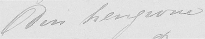Din hengivne
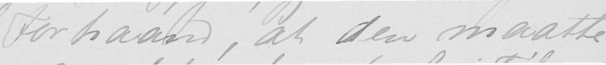Forhaand, at den maatte
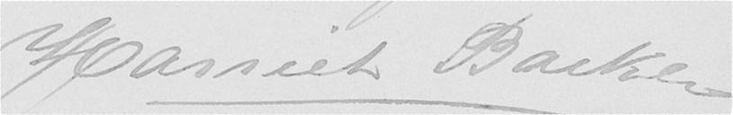Harriet Barker
